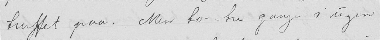truffet paa. Men to-tre gange i ugen
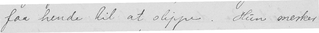faa hende til at slippe. Hun merker
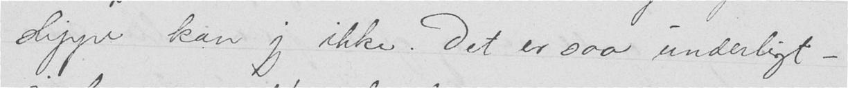slippe kan jeg ikke. Det er saa underligt —
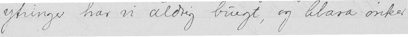ytringer har vi aldrig brugt, og Clara ønsker
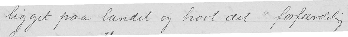ligget paa landet og havt det " forfærdelig
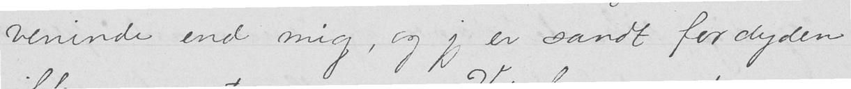veninde end mig, og jeg er sandt for dyden
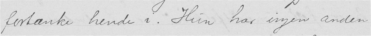fortænke hende i. Hun har ingen anden
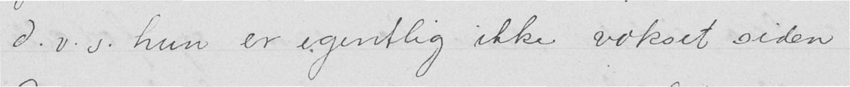d.v.s. hun er egentlig ikke vokset siden.
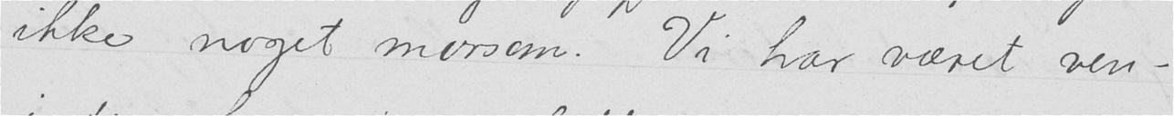ikke noget morsom. Vi har været ven-
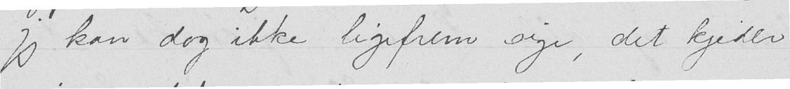jeg kan dog ikke ligefrem sige, det kjeder
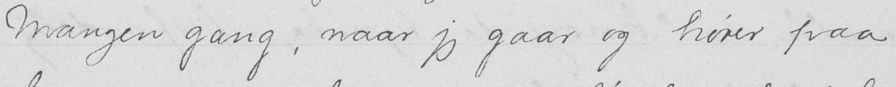Mangen gang, naar jeg gaar og hører paa
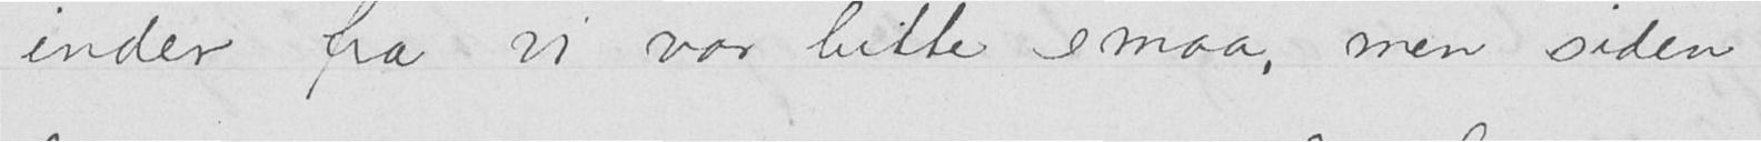inder fra vi var bitte smaa, men siden
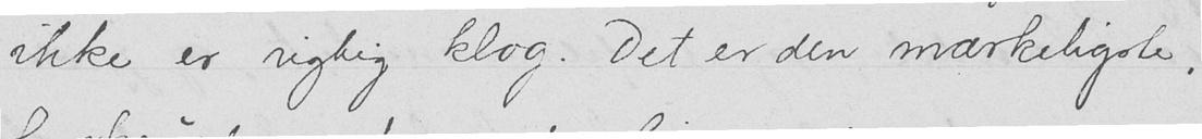ikke er rigtig klog. Det er den mærkeligste,
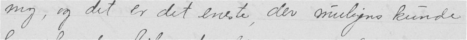mig, og det er det eneste, der muligens kunde
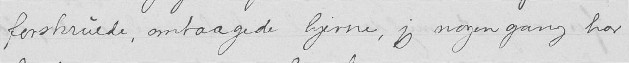forskruede, omtaagede hjerne, jeg nogen gang har
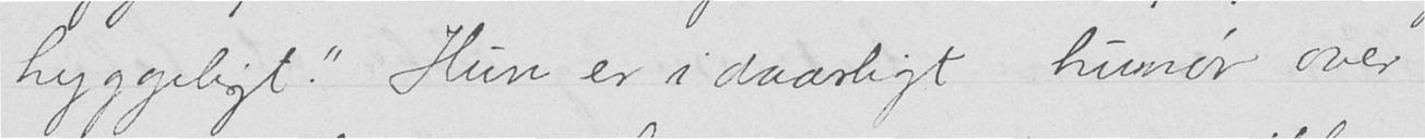hyggeligt." Hun er i daarligt humør over
